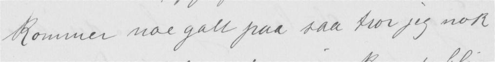kommer noe galt paa saa tror jeg nok
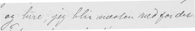og lure, jeg bli næsten ræd for det
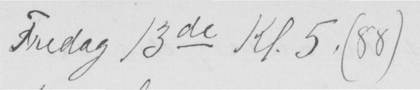Fredag 13de Kl. 5, (88)
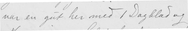var en gut her med 1 Dagblad og
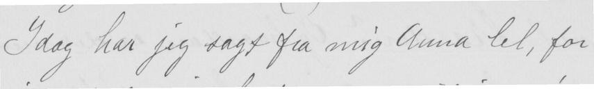Idag har jeg sagt fra mig Anna lel, for
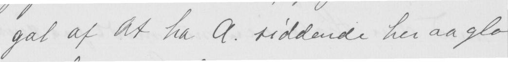gal af at ha A. siddende her aa glo
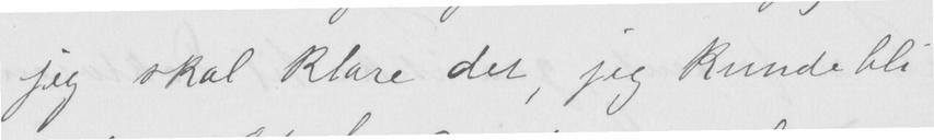jeg skal klare det, jeg kunde bli
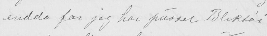endda for jeg har pusset Bliktøi
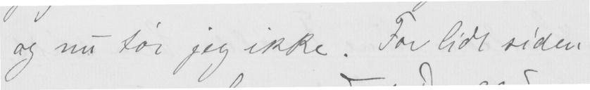og nu tør jeg ikke. For lidt siden
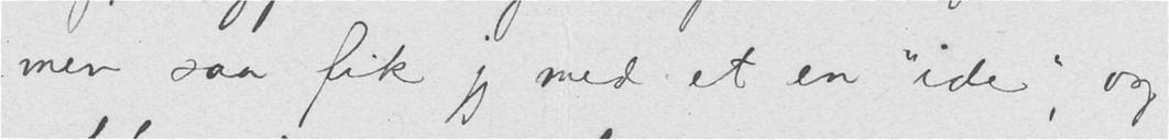men saa fik jeg med et en "ide", og
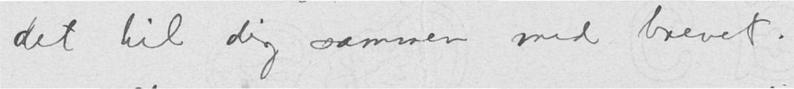det til dig sammen med brevet.
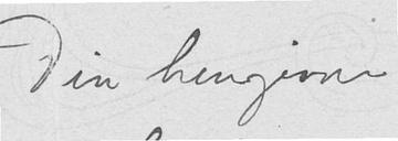Din hengivne
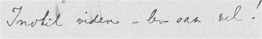Indtil videre — lev saa vel!
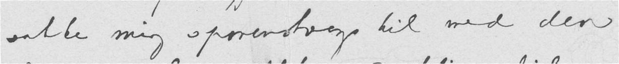satte mig sporenstregs til med den.
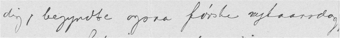dig, begyndte ogsaa første nytaarsdag,
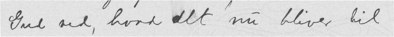Gud ved, hvad det nu bliver til —
